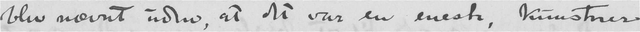ble nævnt uden, at det var en eneste, kunstner.
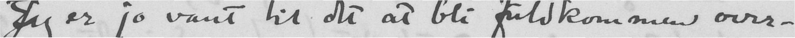Jeg er jo vant til det at bli fuldkommen over-
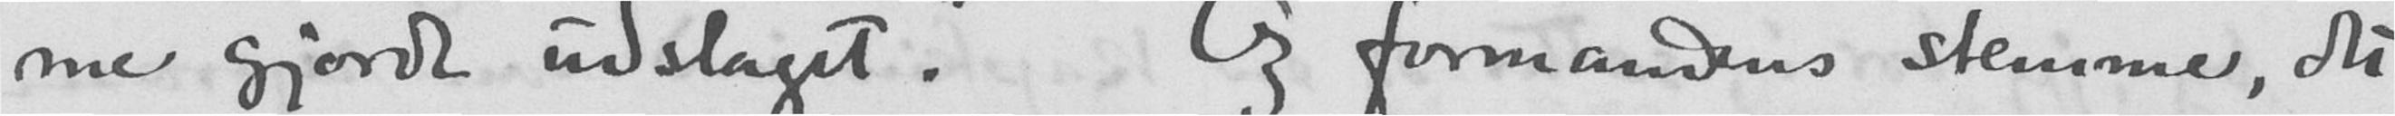me, gjorde udslaget." Og formandens stemme, det
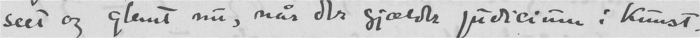seet  og glemt nu, når der gjælder judicium i Kunst-
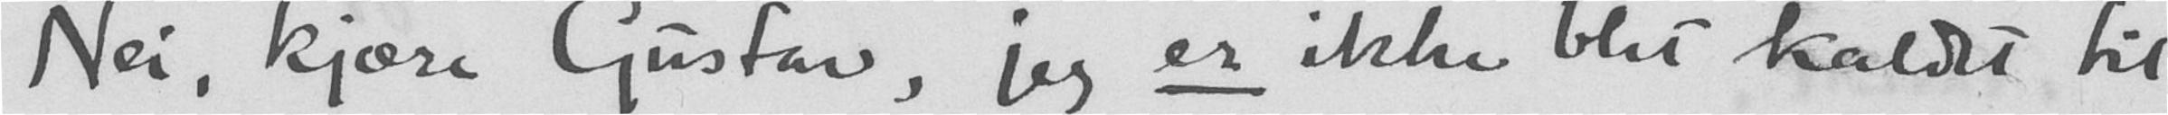Nei, kjære Gustav, jeg er ikke blit kaldt til
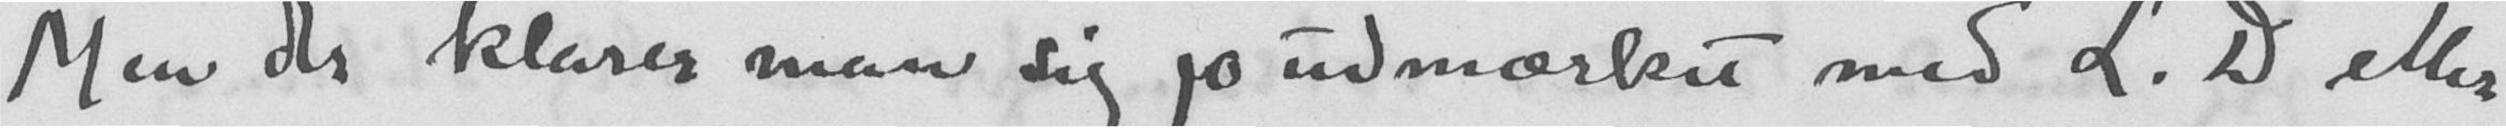Men der klarer man sig jo udmærket med L.D. eller
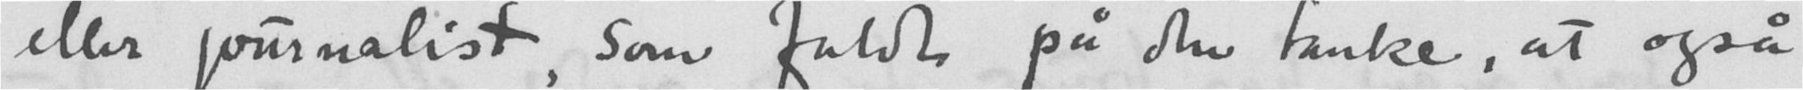eller journalist, som faldt på den tanke, at også
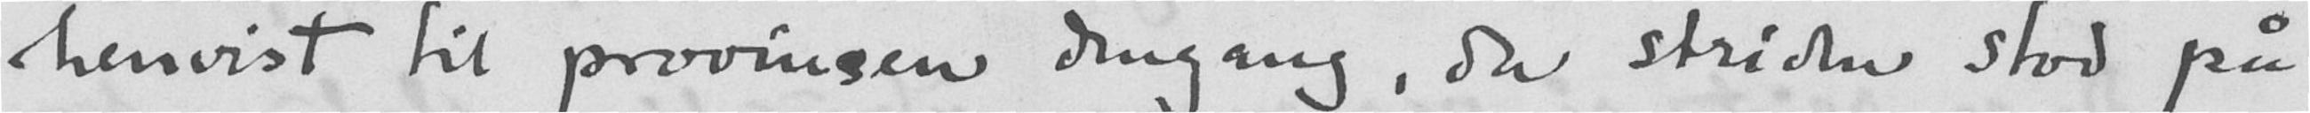henvist til provinsen dengang, da striden stod på
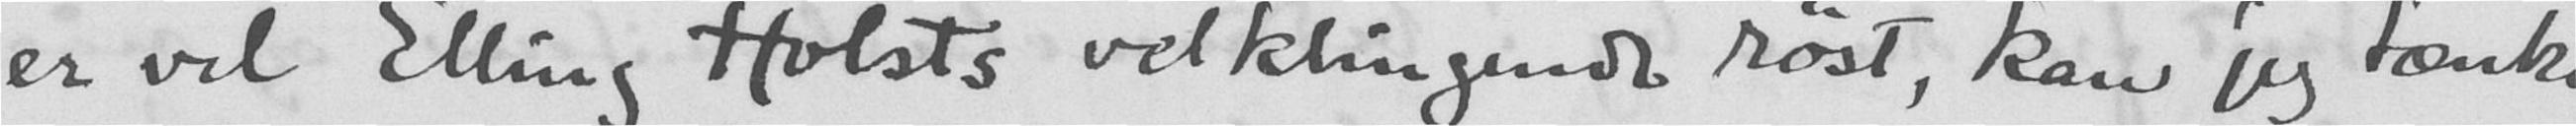er vel Elling Holsts velklingende røst, kan jeg tænke
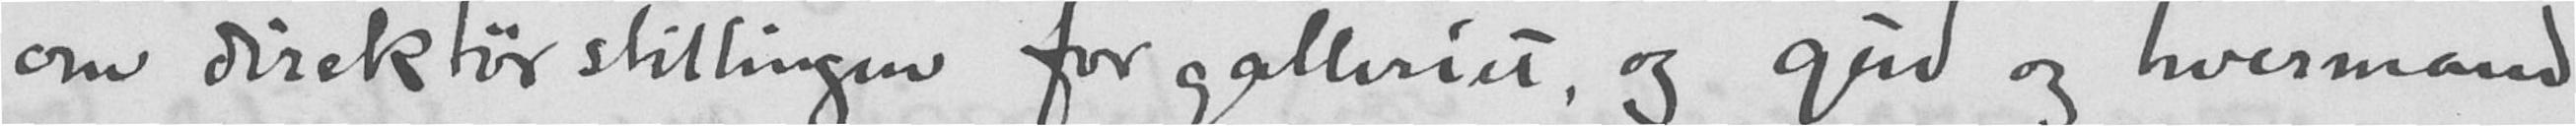om direktørstillingen for galleriet, og gud og hvermand
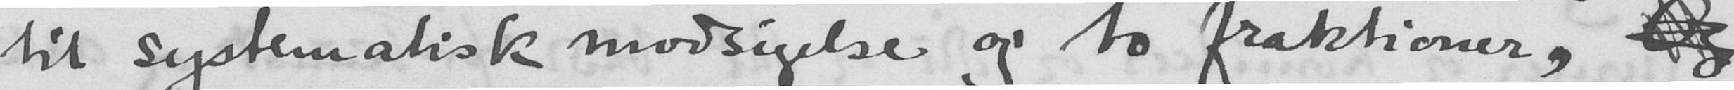til systematisk modsigelse og "to fraktioner, Og
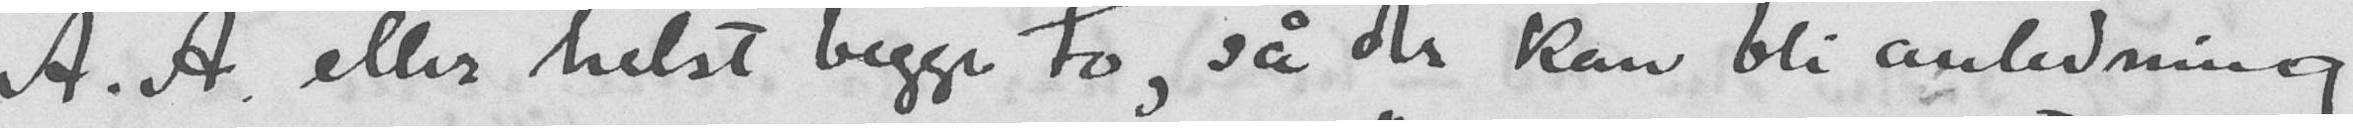A.A. eller helst begge to, så der kan bli anledning
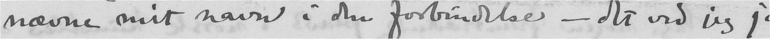nævne mit navn i den forbindelse - det ved jeg jo
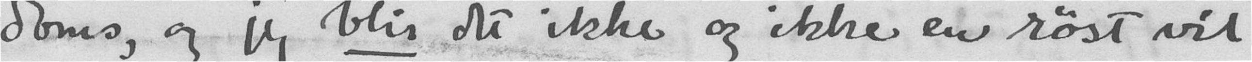doms, og jeg blir det ikke og ikke en røst vil
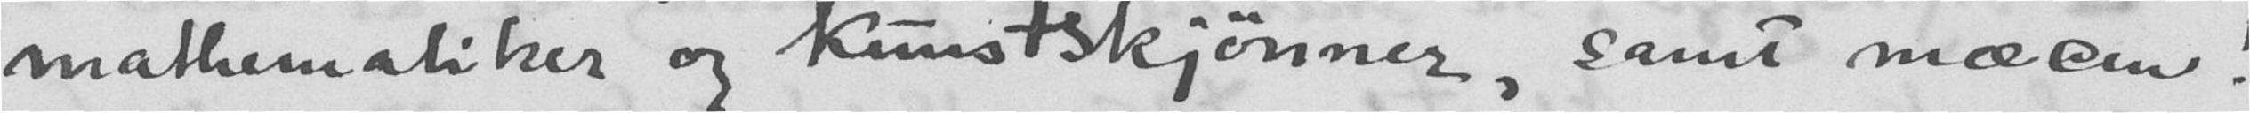mathematiker og kunstskjønner, samt mæcen!
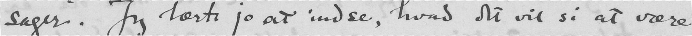sager. Jeg lærte jo at indse, hvad det vil si at være
